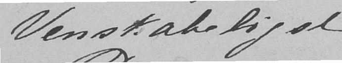Venskabeligst
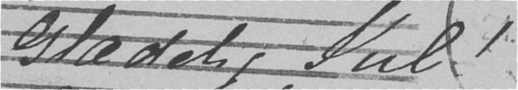Glædelig Jul!
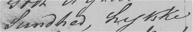Sundhed, Lykke,
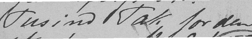Tusind Tak for den
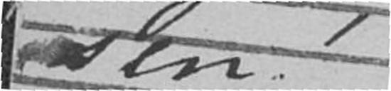sen!
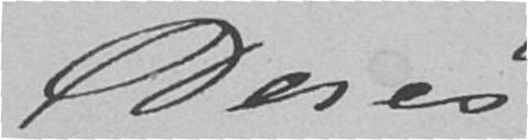Deres
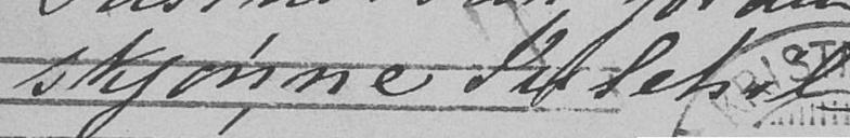skjønne Julehil-
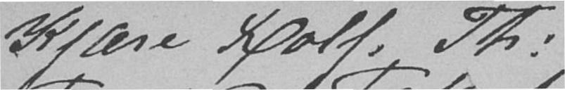Kjære Rolf Th.
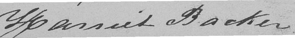Harriet Backer.
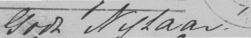Godt Nytaar!
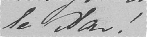le Aar
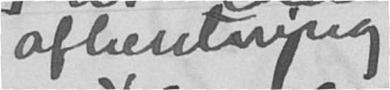afhentning
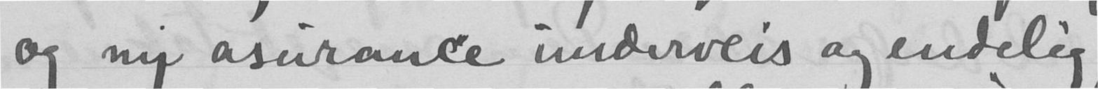og ny asurance underveis og endelig
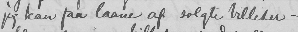jeg kan faa laane af solgte billeder -
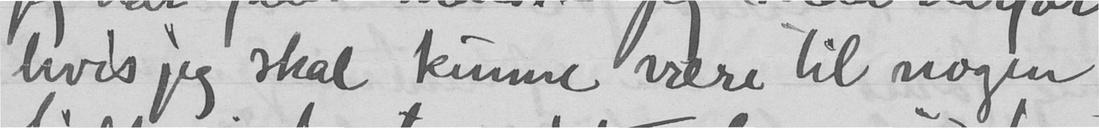hvis jeg skal kunne være til nogen
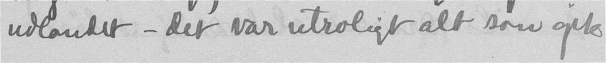udlandet - det var utroligt alt som gik
